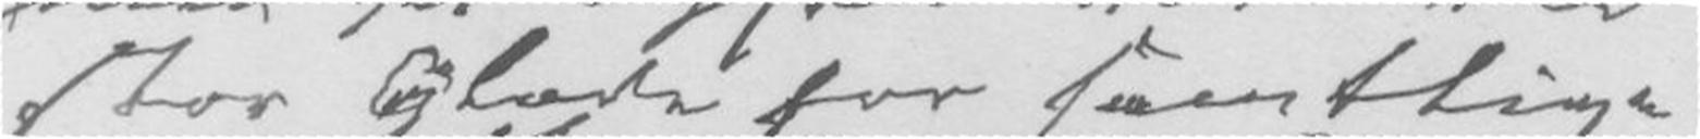stor Glæde for samtlige
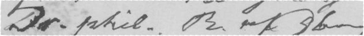Dr. phil. R. af Dborg.
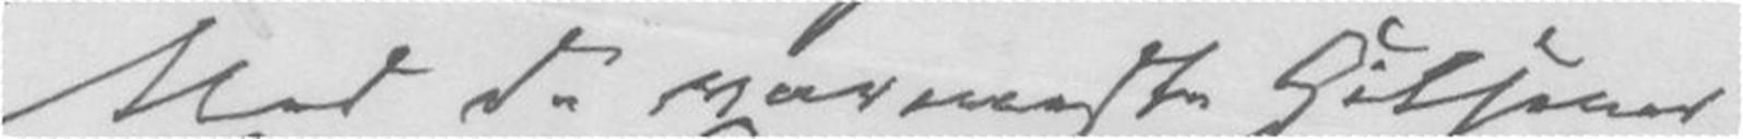Med de varmeste Hilsener
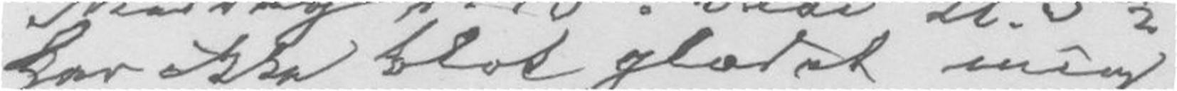har ikke blot glædet mig,
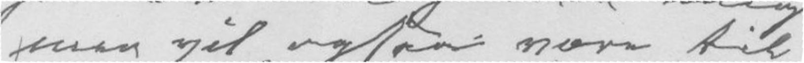men vil ogsaa være til
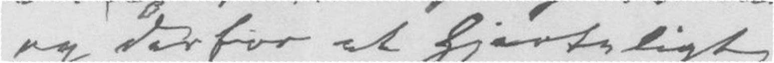og derfor et hjerteligt
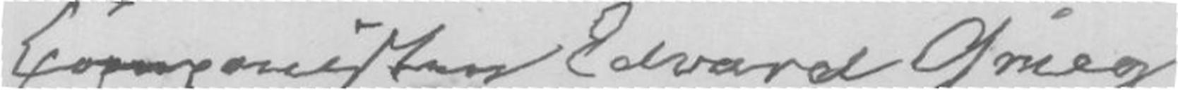Componisten Edvard Grieg
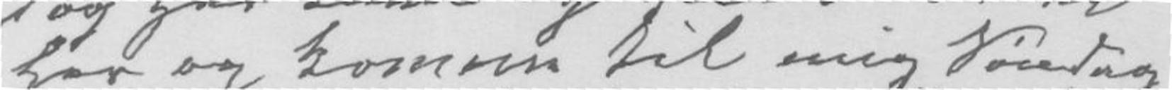her og komme til mig Søndag
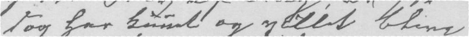dog her kunnet og villet blive
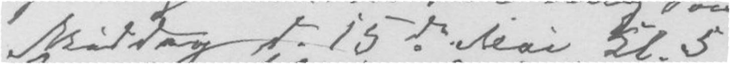Middag d. 15de Mai kl. 5
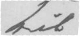Til
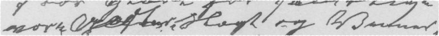vore Gæster, Slægt og Venner,
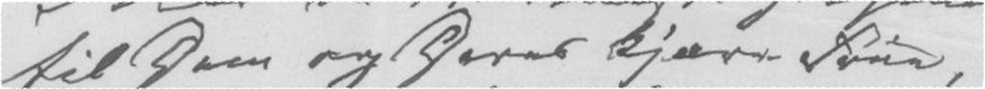til Dem og Deres kjære Frue,
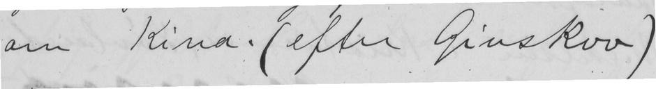om Kina (efter Givskov)
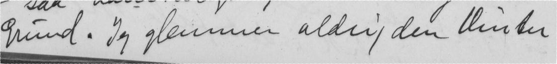Grund. Jeg glemmer aldrig den Vinter
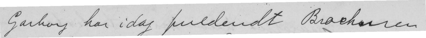Garborg har idag fuldendt Brochuren
